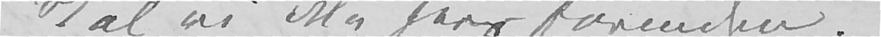Pension.
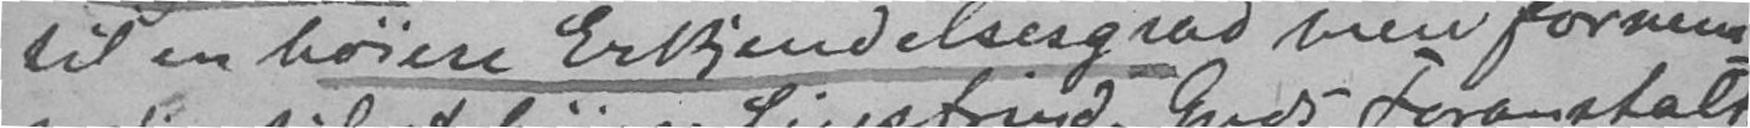til en høiere Erkjendelsesgrad men fornem-
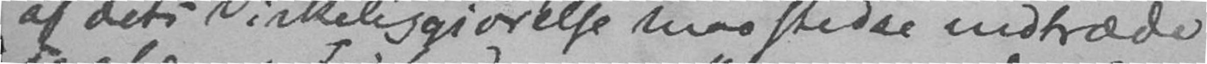af dets Virkeliggjørelse maa stedse indtræde
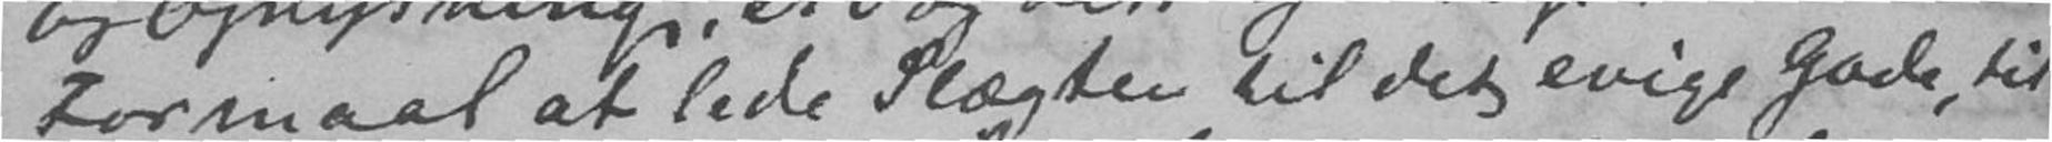Formaal at lede Slægten til det evige Gode, til
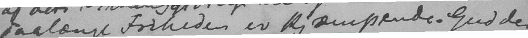saalænge Friheden er kjæmpende. Gud der
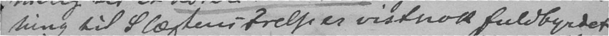ning til Slægtens Frelse er vistnok fuldbyrdet
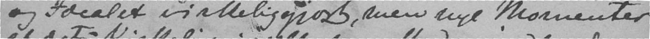og Idealet virikkeliggjort, men nye Momenter
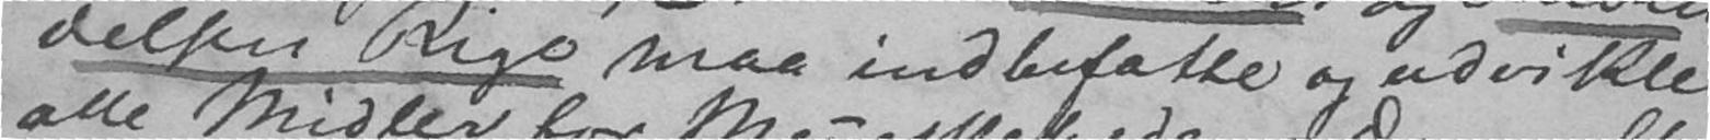delsens Rige maa indbefatte og udvikle
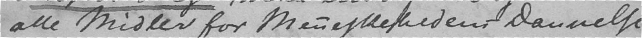alle Midler for Menneskehedens Dannelse
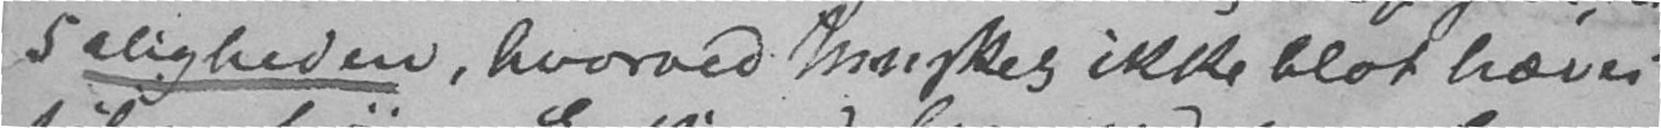Saligheden, hvorved Mennesket ikke blot hæves
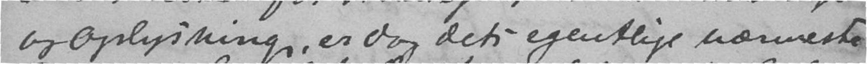og Oplysning, er dog det egentlige nærmeste
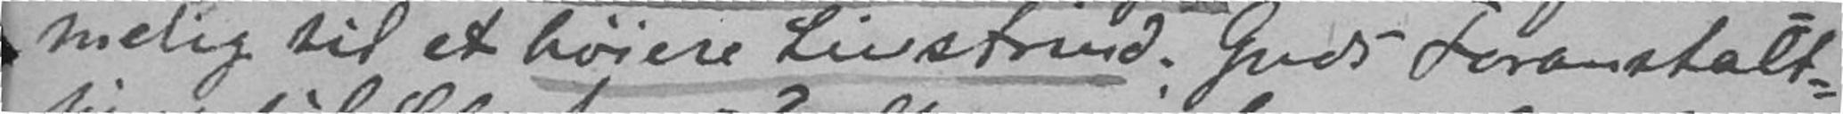melig til et høiere Livstrind. Guds Foranstalt-
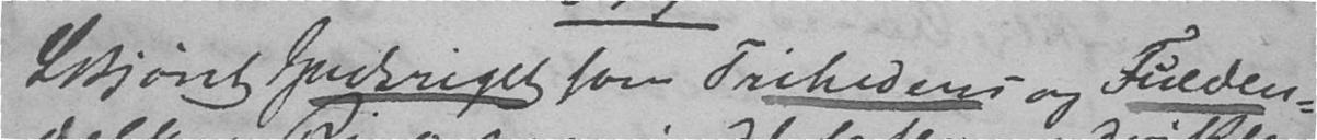Skjønt Gudsriget som Friheden og Fulden-
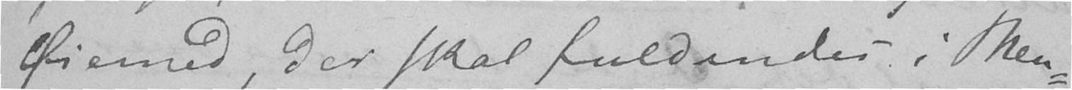Øiemed, der skal fuldendes i Men-
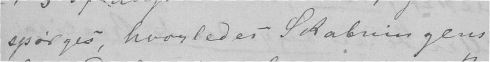spørges, hvorledes Skabningens
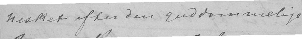nesket efter den guddommelige
